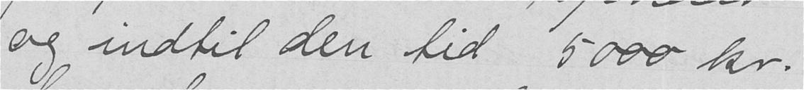og indtil den tid 5000 kr.
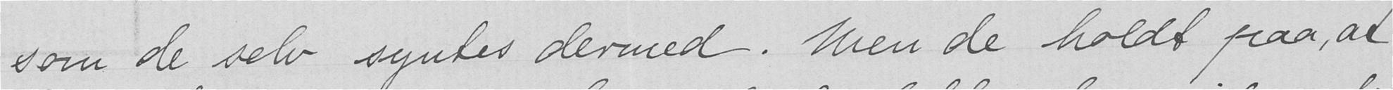som de selv syntes dermed. Men de holdt paa, at
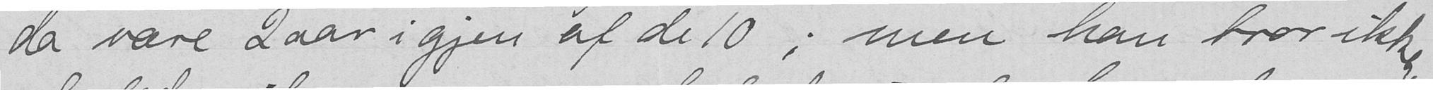da være 2 aar i gjen af de 10; men han tror ikke
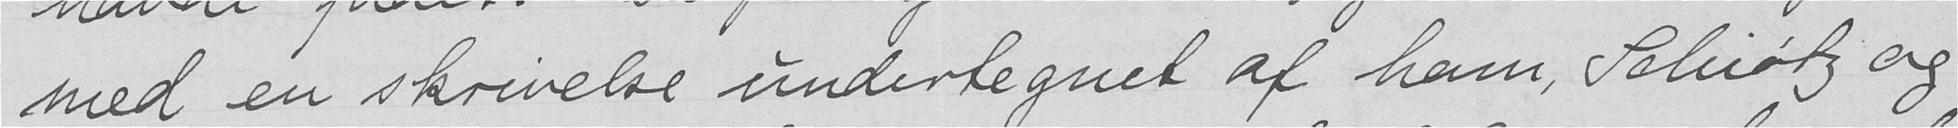med en skrivelse undertegnet at ham, Schiøtz og
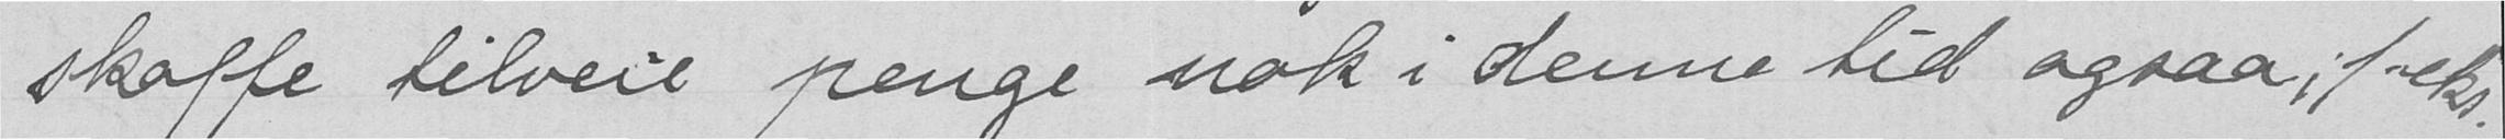skaffe tilveie penge nok i denne tid ogsaa, f.eks
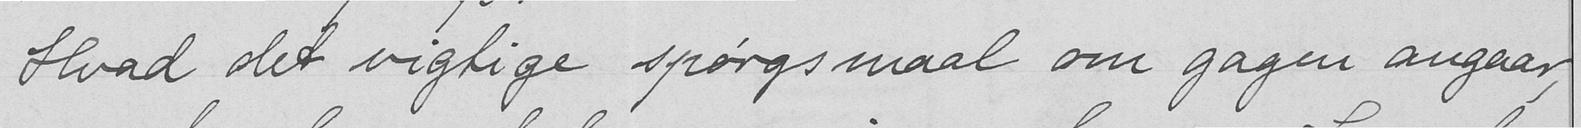Hvad det vigtige spørgsmaal om gagen angaar,
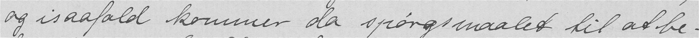og isaafald kommer da spørgsmaalet til at be-
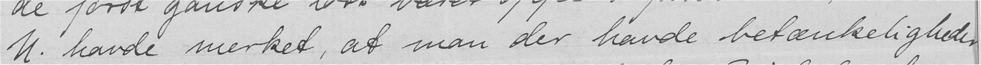N. havde merket, at man der havde betænkeligheder
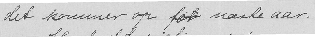det kommer op tat næste aar.
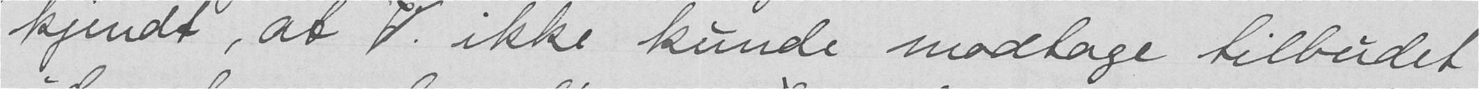kjendt, at V. ikke kunde modtage tilbudet
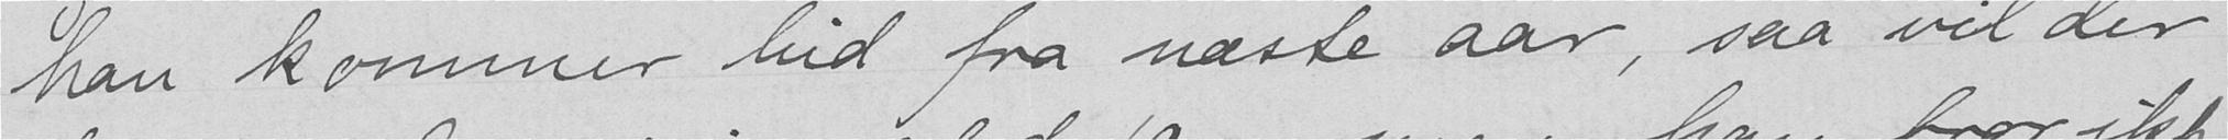han kommer hid fra næste aar, saa vil der
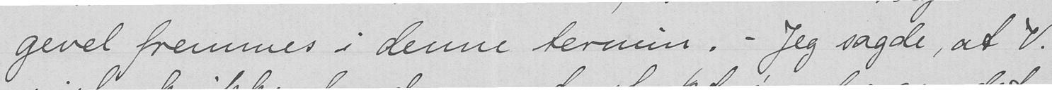gevel fremmes i denne termin. - Jeg sagde, at V.
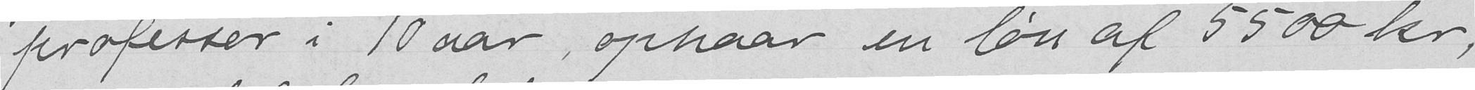professer i 10 aar, opnaar en løn af 5500 kr,
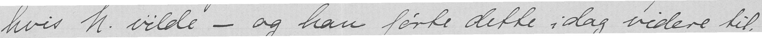hvis N. vilde - og han førte dette idag videre til,
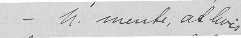- N. mente, at hvis
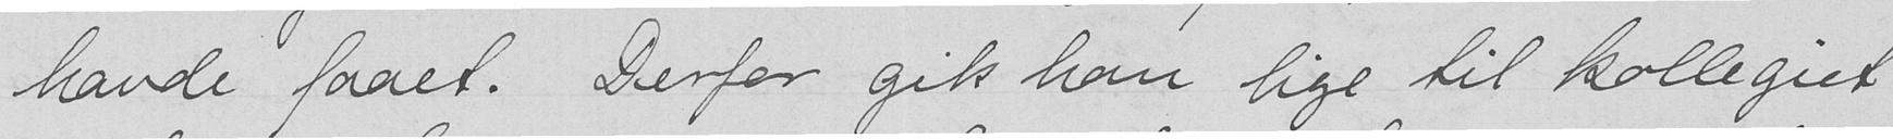havde faaet. Derfor gik han lige til kollegiet
